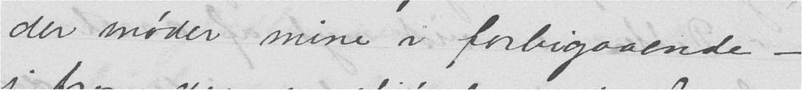der møder mine i forbigaaende -
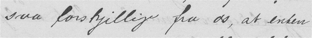saa forskjellig fra os, at enten
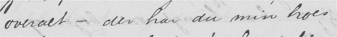overalt - der har du min troes-
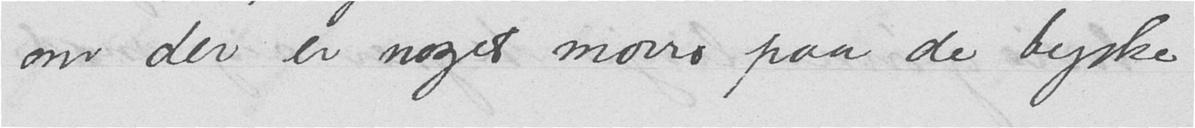om der er noget morro paa de tyske
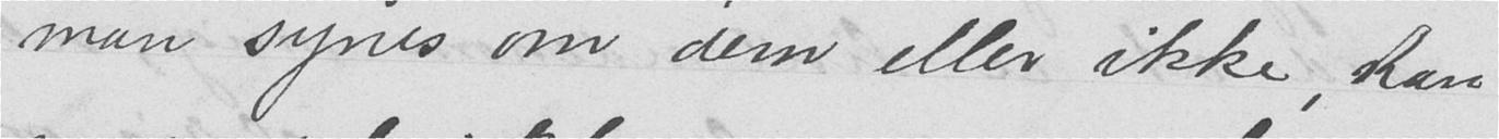man synes om dem eller ikke, kan
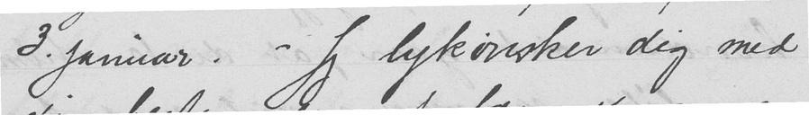3. januar. - Jeg lykønsker dig med
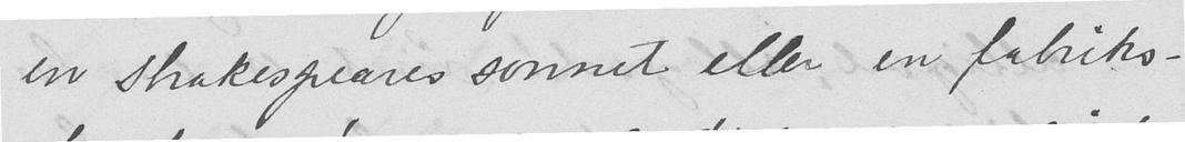en Shakespeares sonnet eller en fabriks-
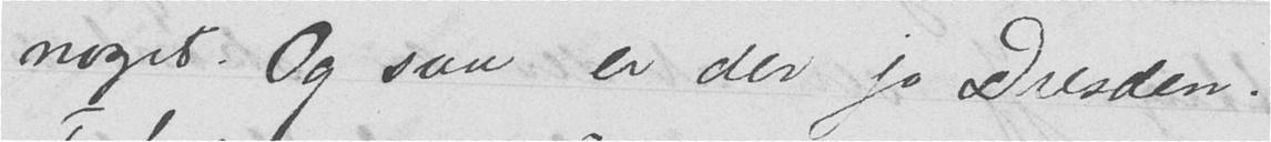noget. Og saa er der jo Dresden.
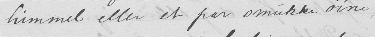himmel eller et par smukke øine
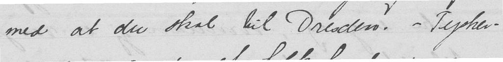med at du skal til Dresden. - Tysker-
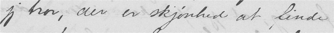jeg tror, der er skjønhed at finde
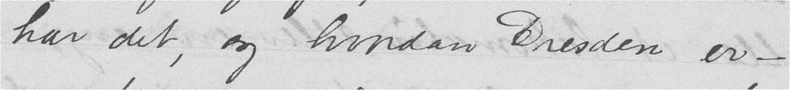har det, og hvordan Dresden er -
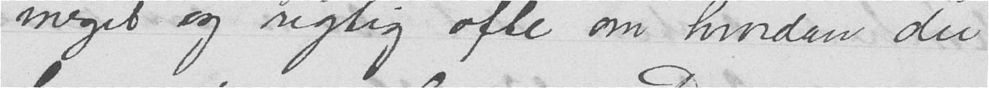meget og rigtig ofte om hvordan du
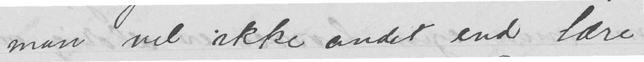man vel ikke andet end lære
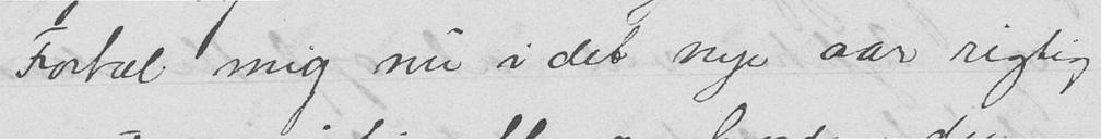Fortæl mig nu i det nye aar rigtig
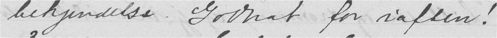bekjendelse. Godnat for iaften!
